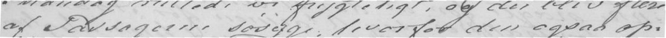af Passagerne søsyge, hvorfor den ogsaa op-
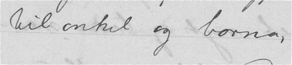til onkel og barna,
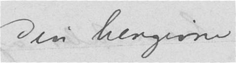Din hengivne
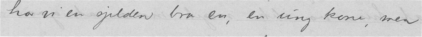har vi en sjelden bra en, en ung kone, men
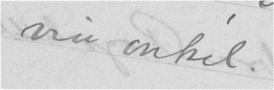via onkel.
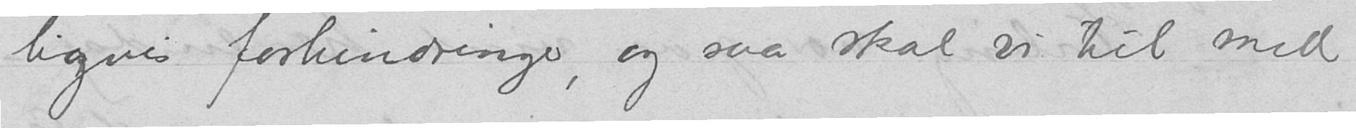ligvis forhindringer, og saa skal vi til med
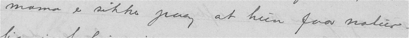mama er sikker paa at hun faar natur-
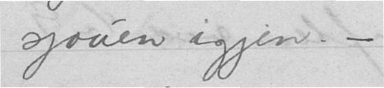sjouen igjen. –
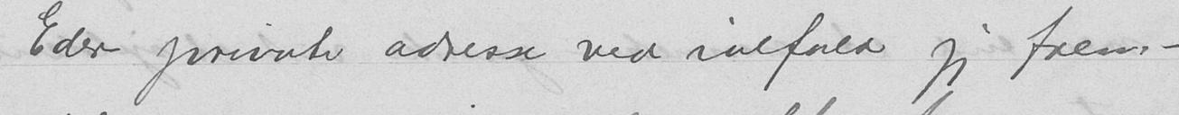Eders private adresse ved ialfald jeg frem-
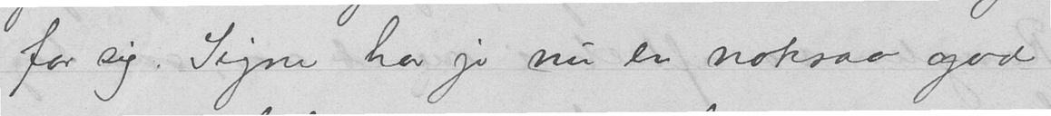for sig. Signe har jo nu er noksaa god
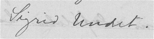Sigrid Undset.
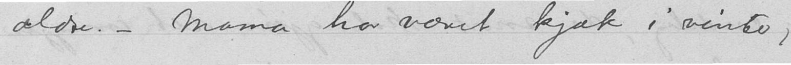ældre. – Mama har været kjæk i vinter,
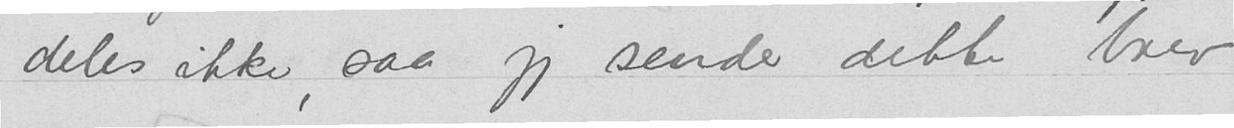deles ikke, saa jeg sender dette brev
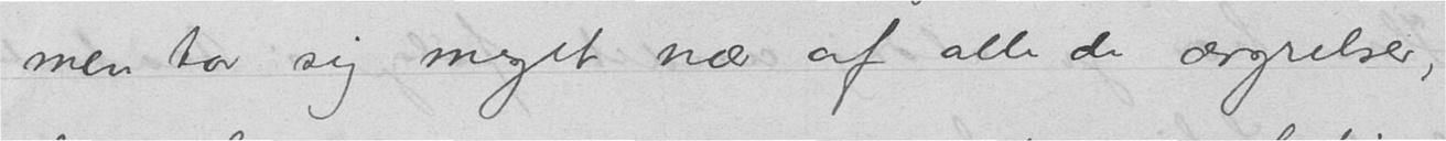men tar sig meget nær af alle de ærgrelser,
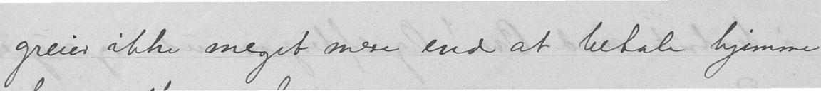greier ikke meget mere end at betale hjemme
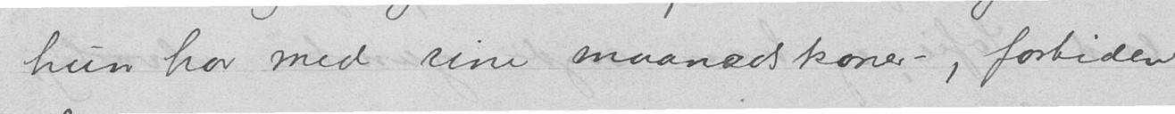hun har med sine maanedskoner –, fortiden
